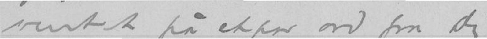ventet på etpar ord fra dig
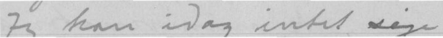Jeg kan idag intet sige
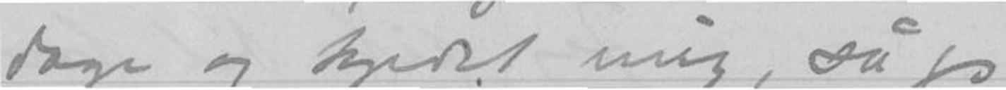dage og kjedet mig, så jeg
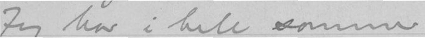Jeg har i hele sommer
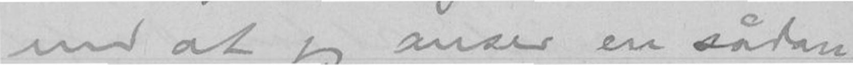end at jeg anser en sådan
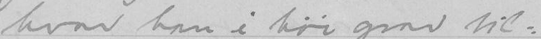hvad han i høi grad til-
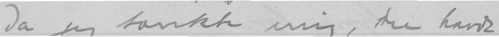Ja jeg tænkte mig, de havde
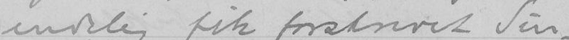endelig fik forskrevet Sin-
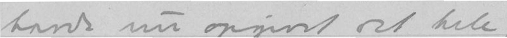havde nu opgivet det hele,
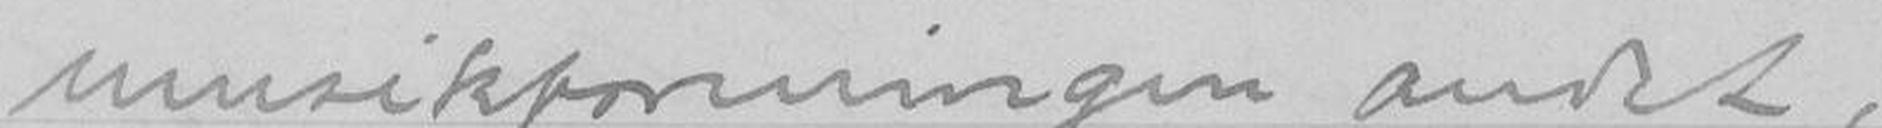musikforeningen andet,
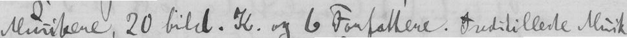Musikere, 20 bild. K. og 6 Forfattere. Indstillede Musik.
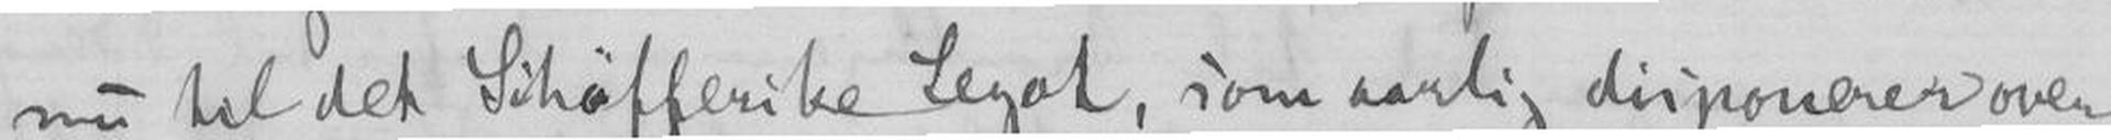nu til det Schäfferike Legat, som aarlig disponerer over
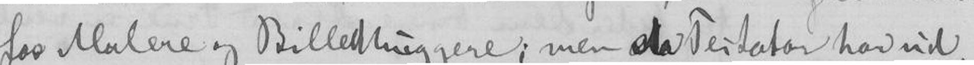for Malere og Billedhuggere; men da Testator har ud-
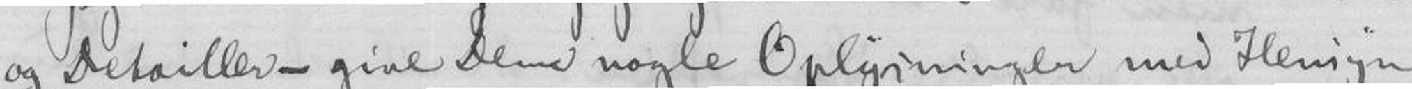og Detailler - give Dem nogle Oplysninger med Hensyn
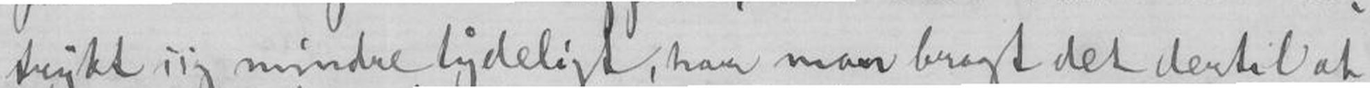trykt sig mindre tydeligt, har man brugt det dertil at
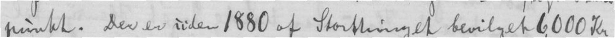punkt. Der er siden 1880 af Storthinget bevilget 6000 Kr.
